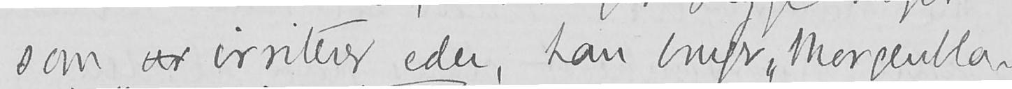som vr irriterer eder, han bruger "Morgenbla-
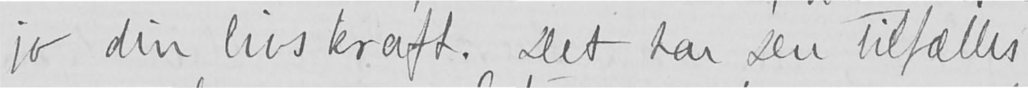jo din livskraft. Det har Du tilfælles
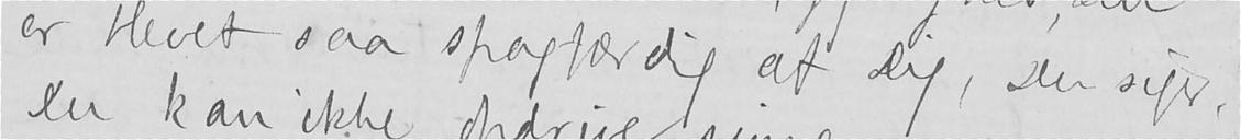er blevet saa spagfærdig af Dig, Du siger,
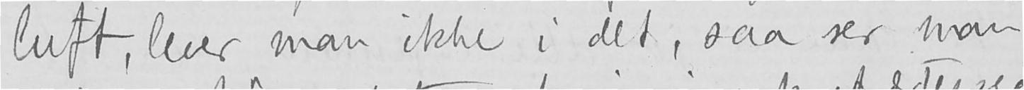luft, lever man ikke i det, saa ser man
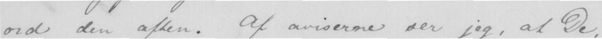ord den aften. Af aviserne ser jeg, at De,
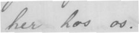her hos os.
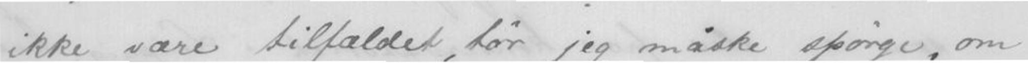ikke være tilfældet, tør jeg måske spørge, om
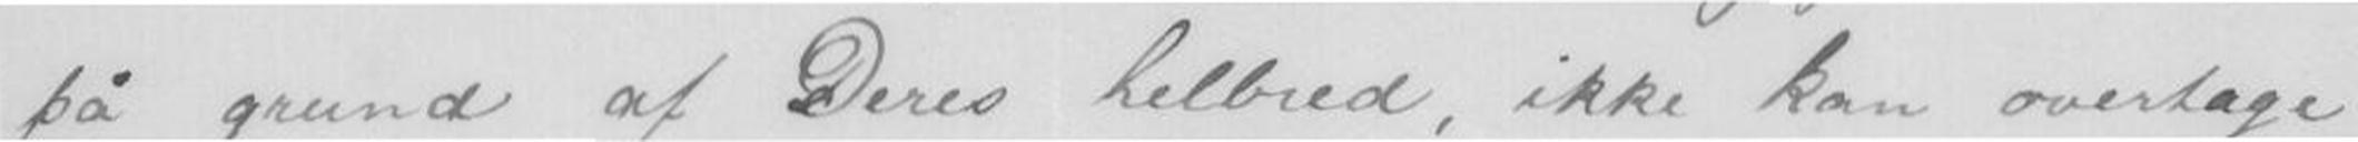på grund af Deres helbred, ikke kan overtage
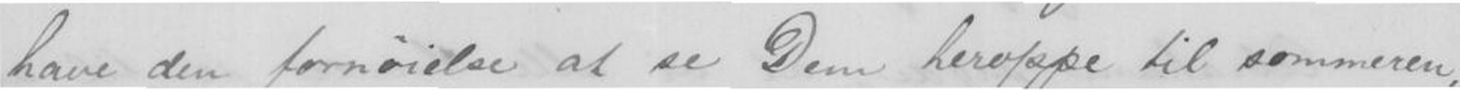have den fornøielse at se Dem heroppe til sommeren,
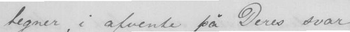tegner, i afvente på Deres svar,
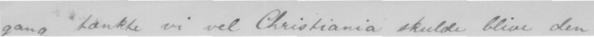gang tænkte vi vel Christiania skulde blive den
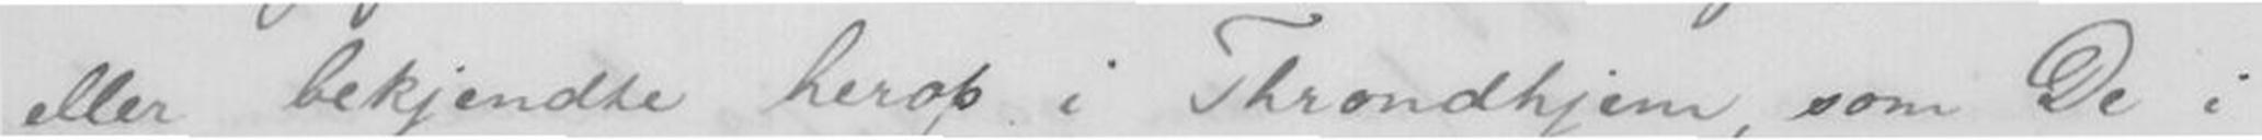eller bekjente herop i Trondhjem, som De i
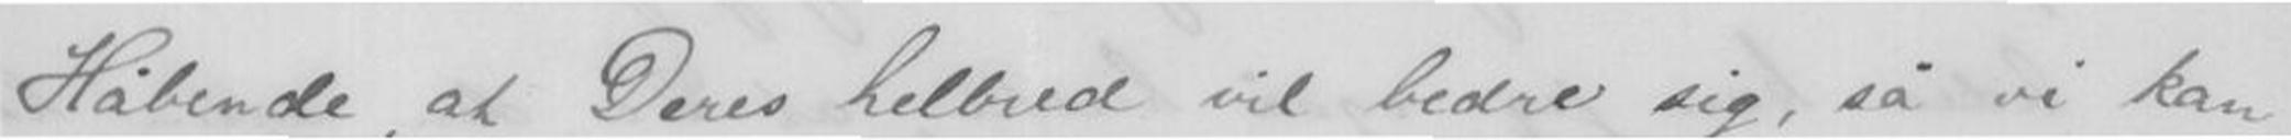Håbende, at Deres helbred vil bedre sig, så vi kan
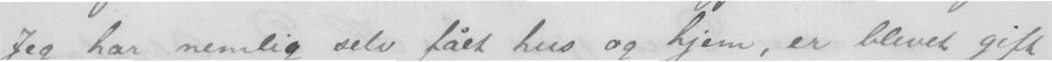Jeg har nemlig selv fået hus og hjem, er blevet gift
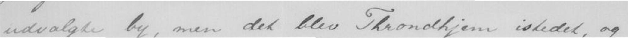udvalgte by, men det blev Trondhjem istedet, og
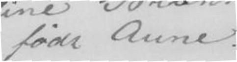født Aune.
